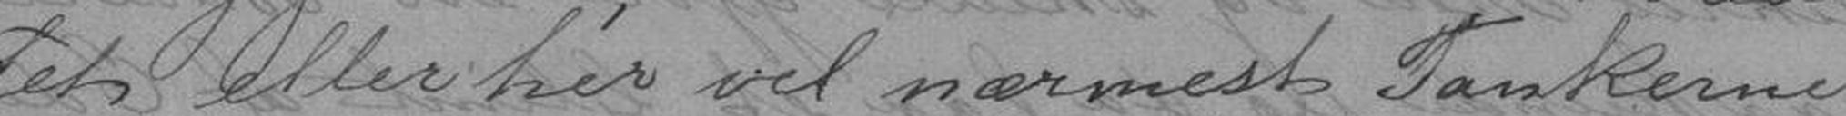tet eller her vel nærmest Tankerne er
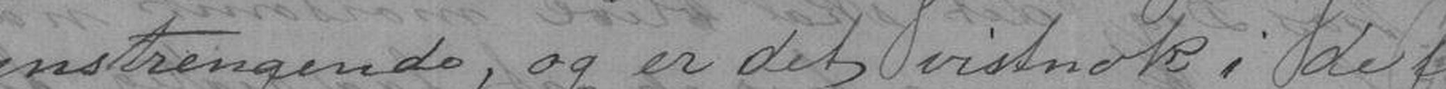anstrengende, og er det vistnok i de fle-
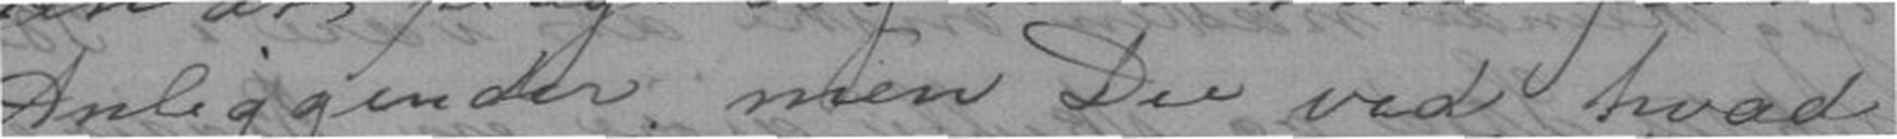Anliggender; men Du ved hvad
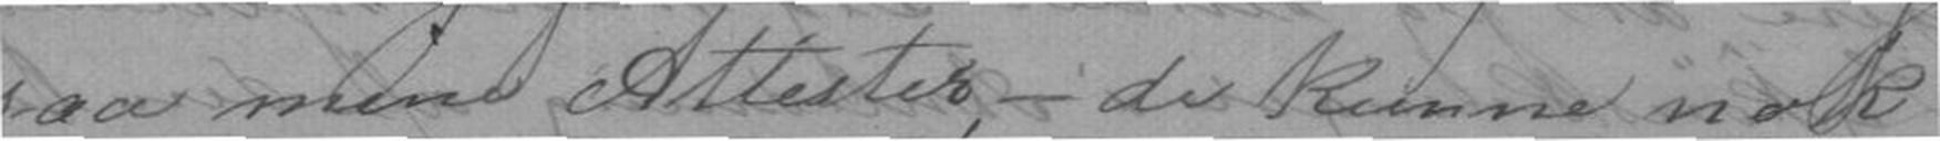saa mine Attester, - de kunne nok
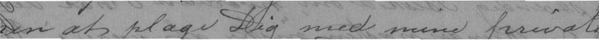gen at plage Dig med mine private
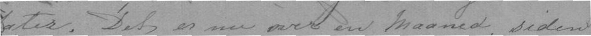tater. Det er nu en Maaned siden
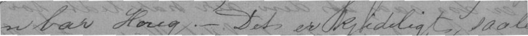en bar Haug. - Det er kjedeligt, saal
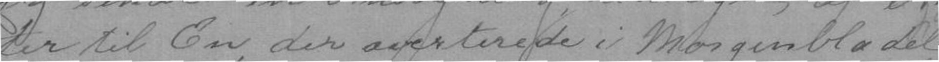ter til En, der averterede i Morgenbladet
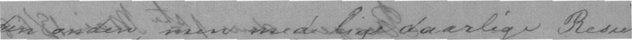den anden, men med lige daarlige Resul-
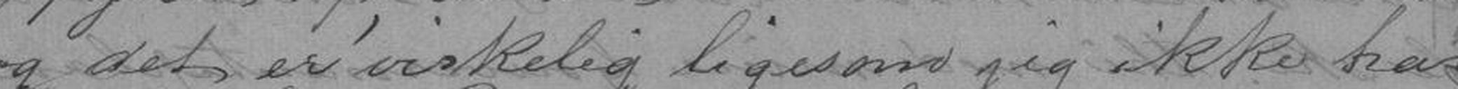og det er virkelig ligesom jeg ikke har
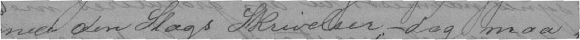med den Slags Skrivelser, - dog maa
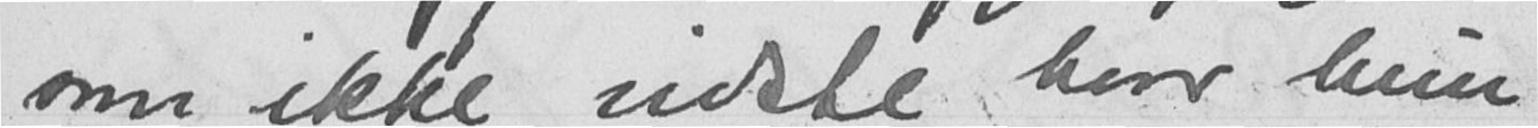som ikke vidste hvor hun
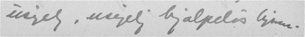usigelig, usigelig hjælpeløs ligsom.
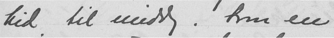hid til middag. Som en
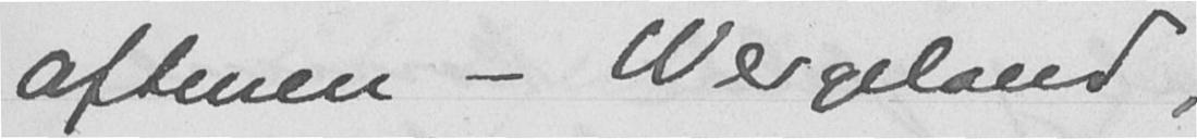aftenen - Wergeland,
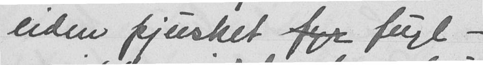liden pjusket fyr fugl -
